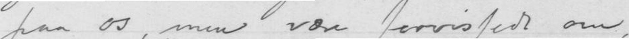paa os, men være forvisedt om,
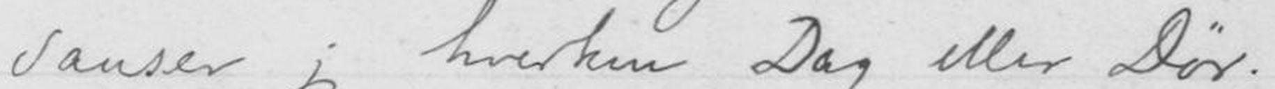sanser jeg hverken Dag eller Død.
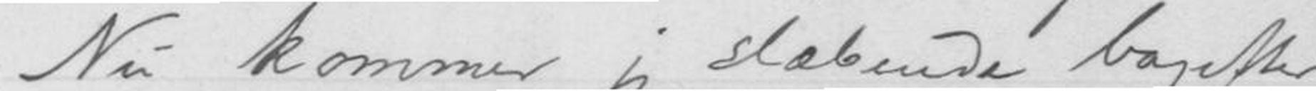Nu kommer jeg slæbende bagefter
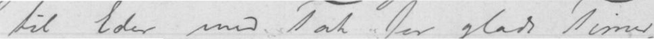til Eder med Tak for glade Timer
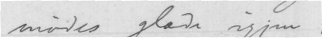mødes glade igjen.
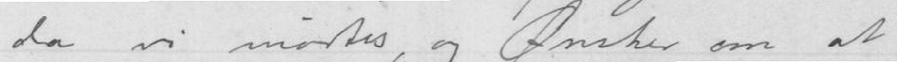da vi mødtes, og Ønsker om at
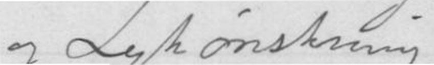og Lykønskning
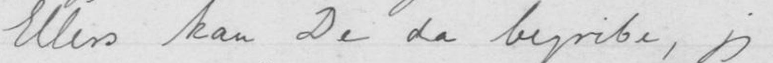Ellers kan De da begribe, jeg
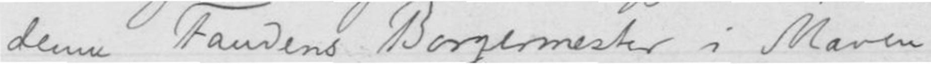denne Fandens Borgermester i Maven
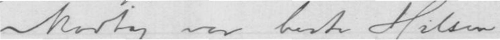vor bedste Hilsen
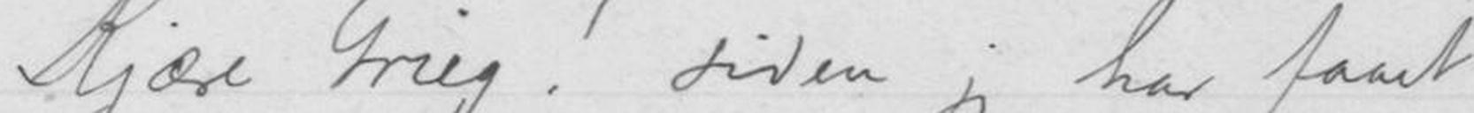Kjære Grieg! Siden jeg har faaet
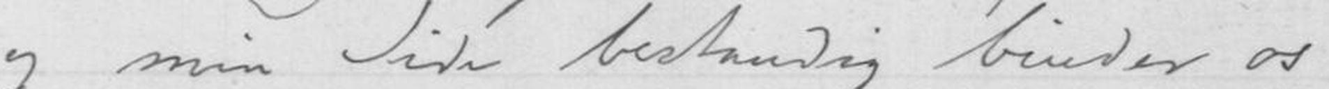og min side bestandig binder os
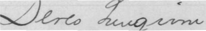Deres hengivne
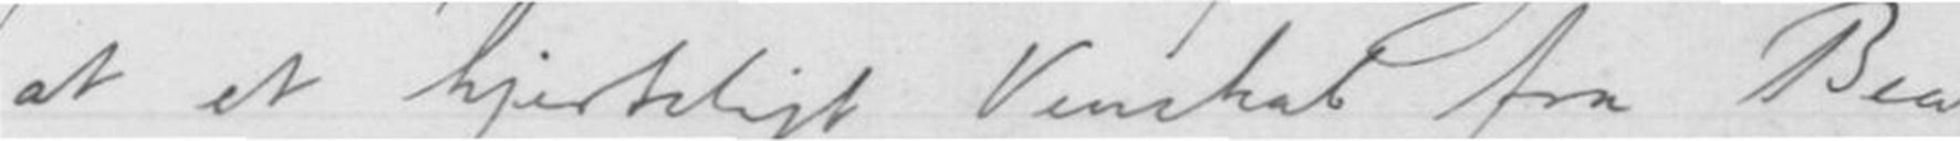at et hjerteligt Venskab fra Bea
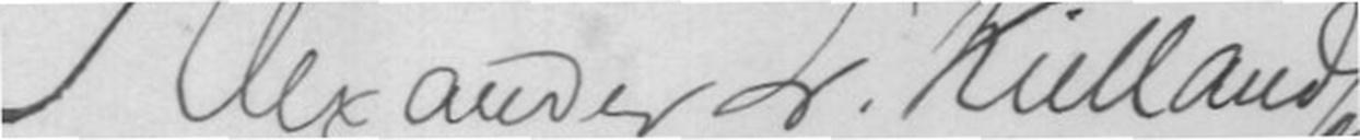Alexander L. Kielland
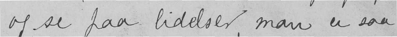og se paa lidelser, man er saa
